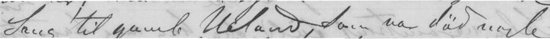Sang til gamle Ueland, som var død nogle
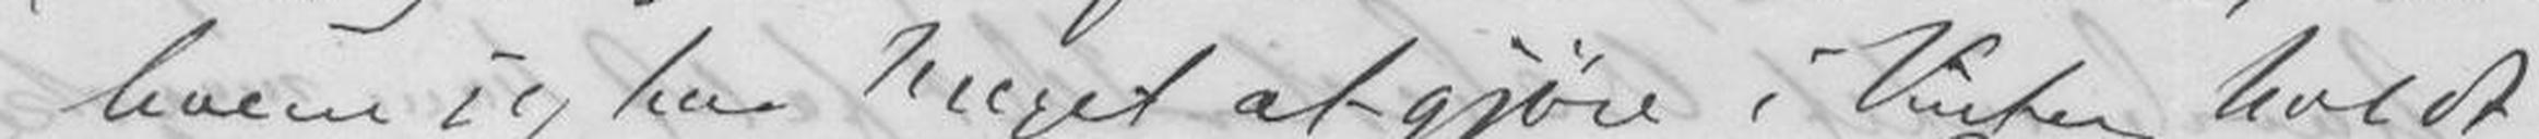hvem jeg har Meget at gjøre i Vinter, holdt
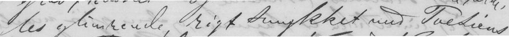les glimrende, rigt smykket med Poesiens
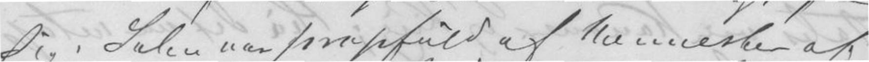sig; Salen var propfuld af Mennesker af
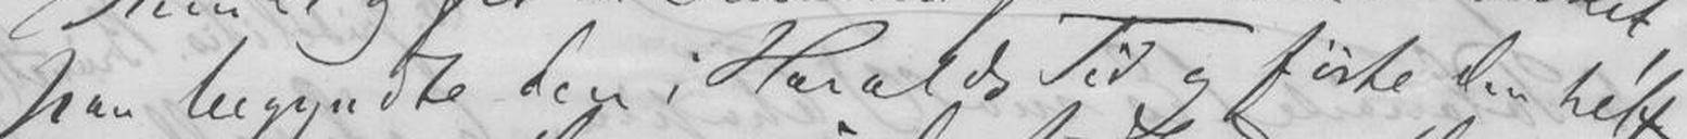han begyndte den i Haralds Tid og førte den helt
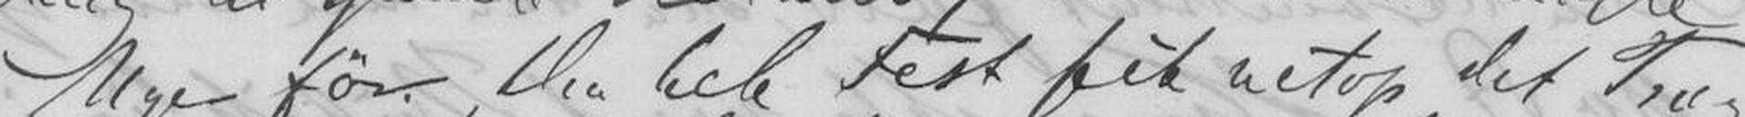Uge før. Den hele Fest fik netop det Præg,
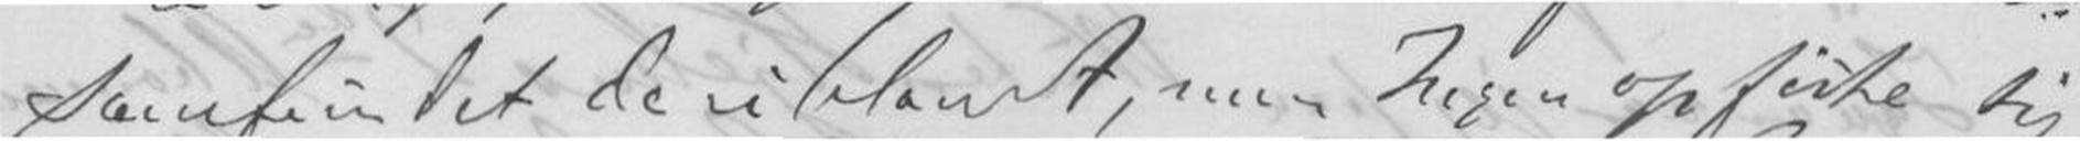samfundet deriblandt, men Ingen opførte sig
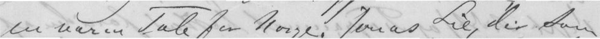en varm Tale for Norge, Jonas Lie, der som
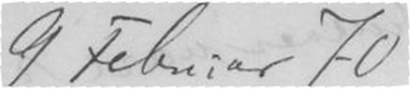9 Februar 70.
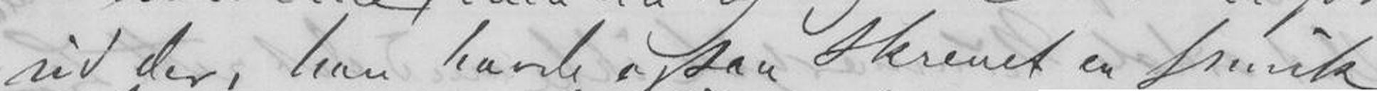ud der; han havde ogsaa skrevet en smuk
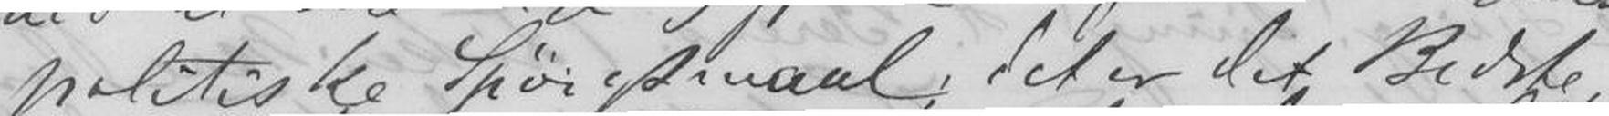politiske Spørgsmaal; det er det Bedste,
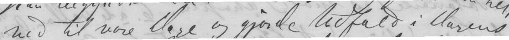ned til vore Dage og gjorde Udfald i Dagens
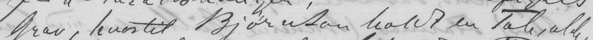Grav, hvortil Bjørnson holdt en Tale, alde-
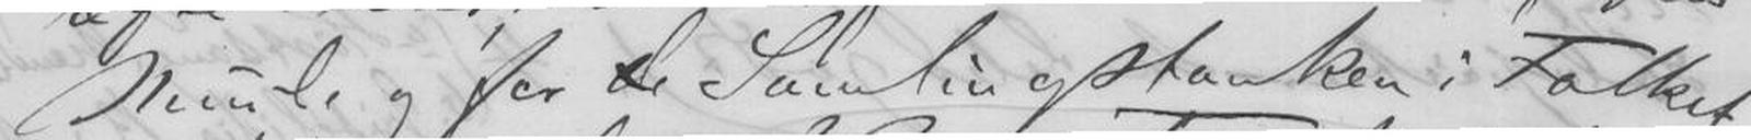Minde og for de Samlingstanken i Folket,
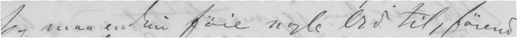Jeg maa endnu føie nogle Ord til, førend
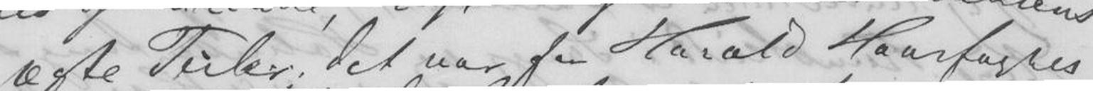ægte Perler; det var for Harald Haarfagres
